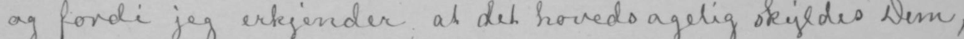og fordi jeg erkjender at det hovedsagelig skyldes Dem,
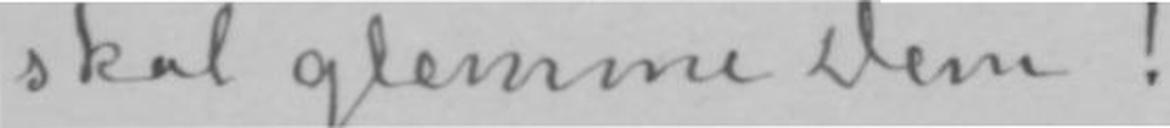skal glemme Dem!
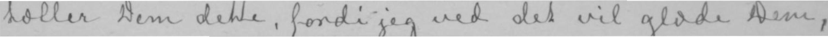tæller Dem dette, fordi jeg ved det vil glæde Dem,
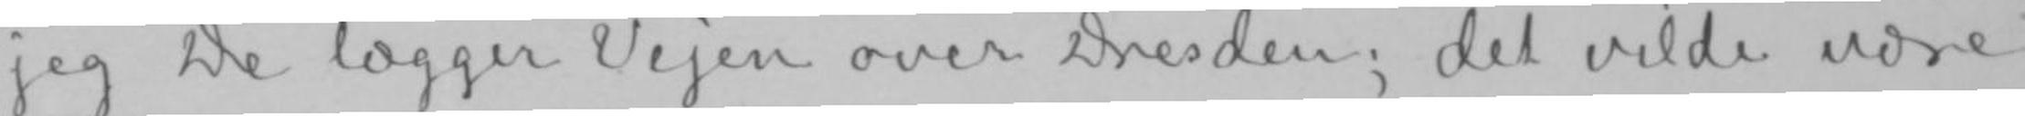jeg De lægger Vejen over Dresden; det vilde være
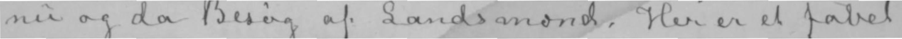nu og da Besøg af Landsmænd. Her er et fabel-
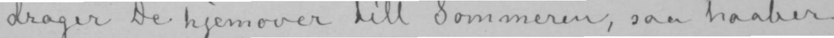drager De hjemover till Sommeren, saa haaber
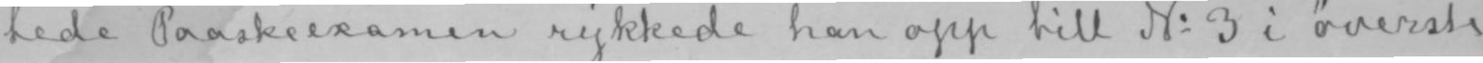tede Paaskeexamen rykkede han opp till No 3 i øverste
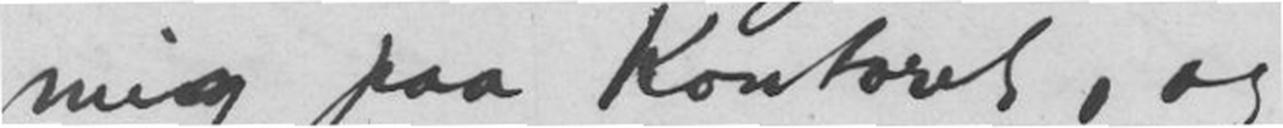mig paa Kontoret, og
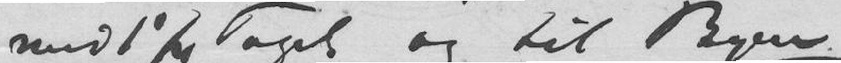med 1 ½ Toget og til Byen
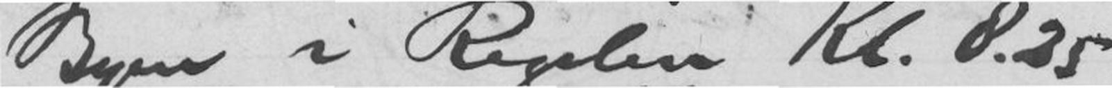Byen i Regelen Kl. 8.25
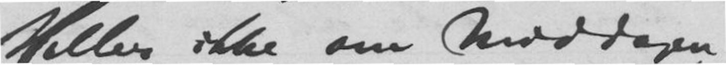Heller ikke om Middagen
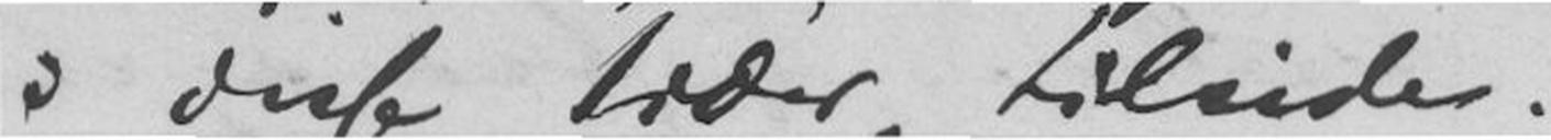i disse Tider, tilside.
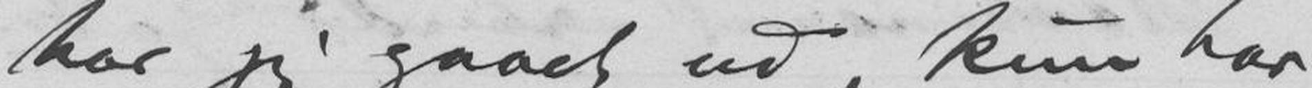har jeg gaaet ud, kun har
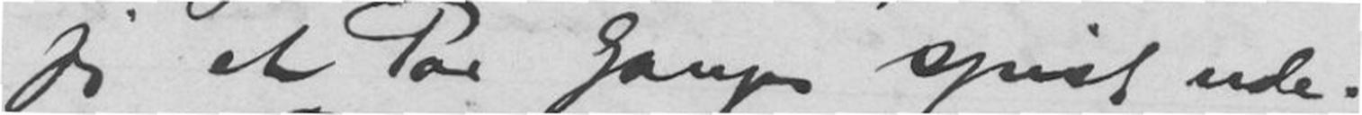jeg et Par Gange spist ude.
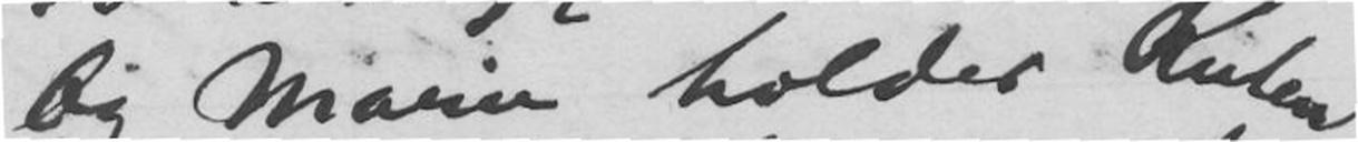Og Marie holder Ruten
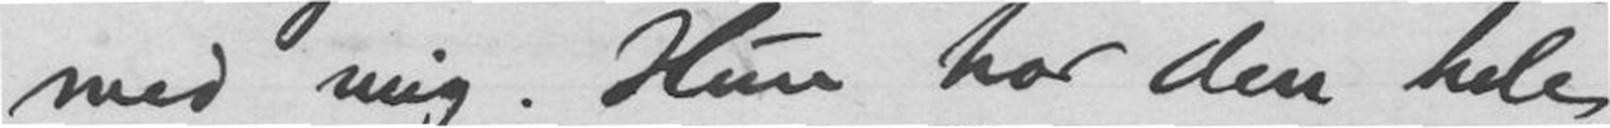med mig. Hun har den hele
